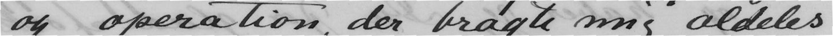og operation, der bragte mig aldeles
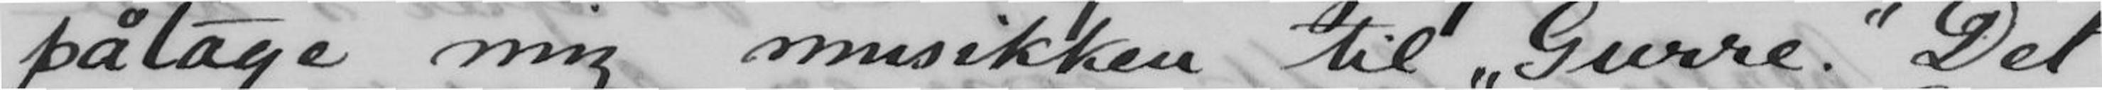påtage mig musikken til Gurre. Det
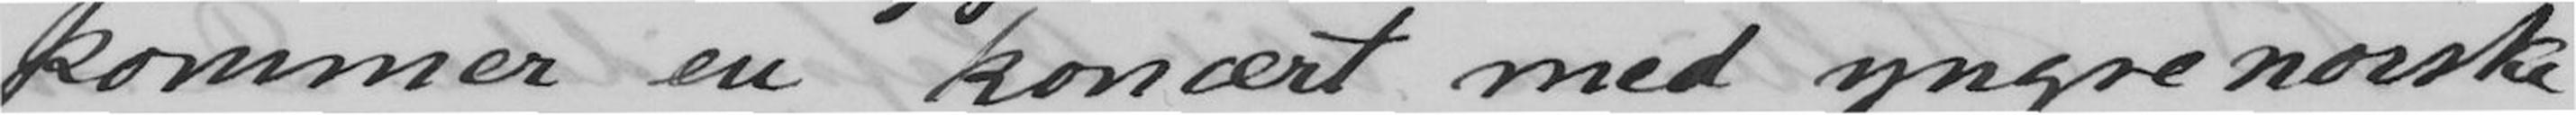kommer en koncert med yngre norske
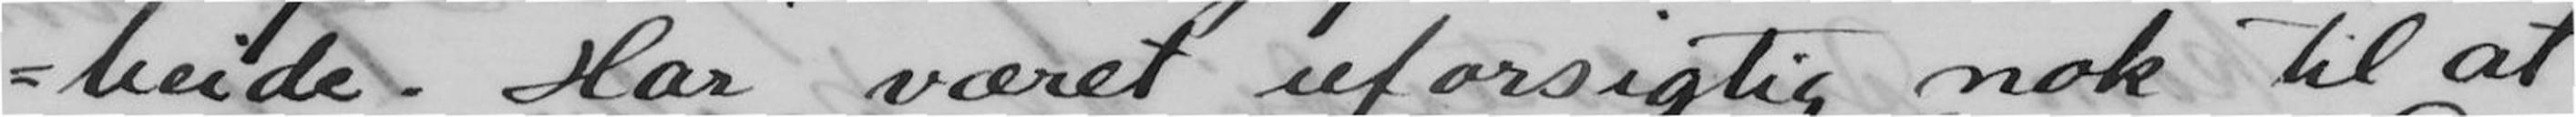beide. Har været uforsigtig nok til at
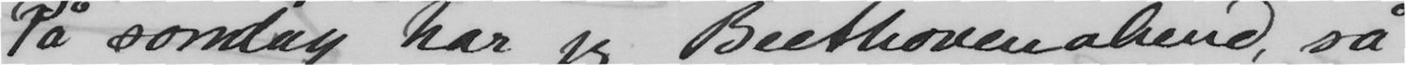På søndag har jeg Beethovenabend, så
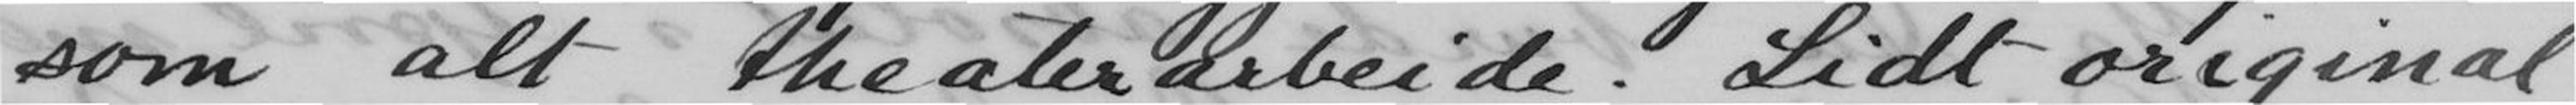som alt theaterarbeide. Lidt original
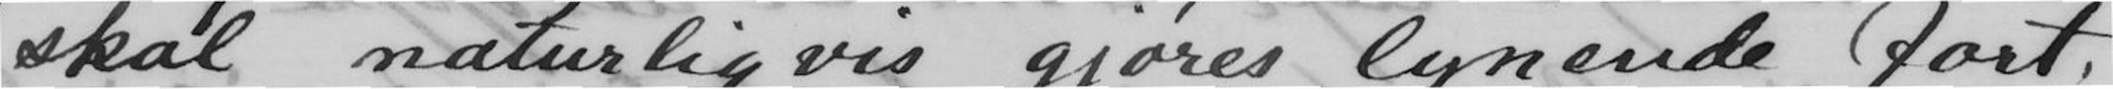skal naturligvis gjøres lynende fort,
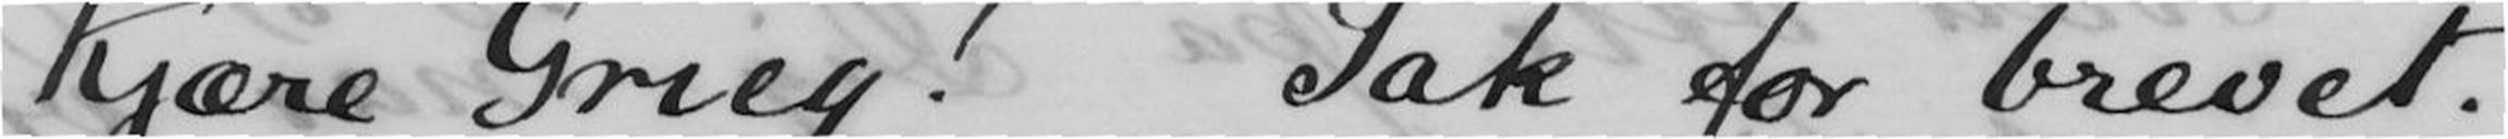Kjære Grieg! Tak for brevet.
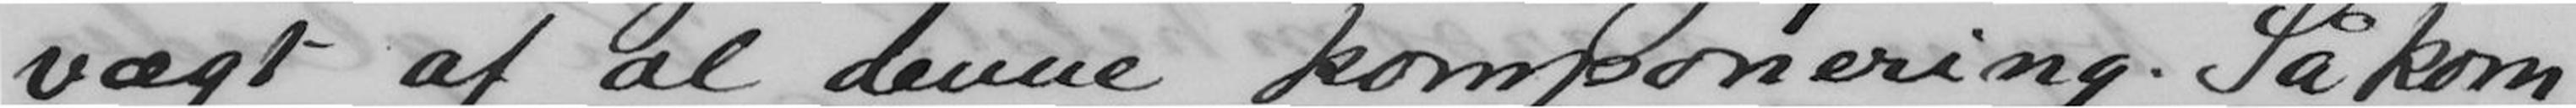vægt af al denne komponering. Så kom-
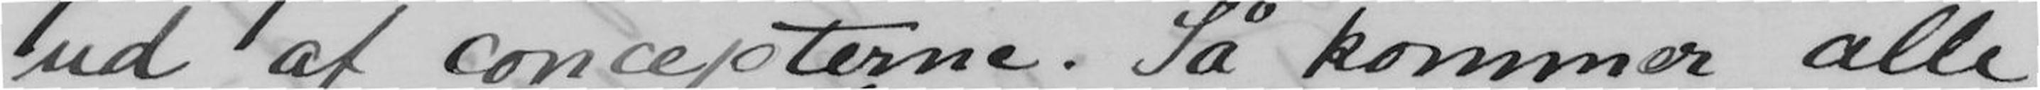ud af concepterne. Så kommer alle
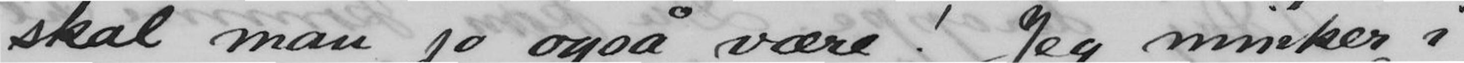skal man jo også være! Jeg minker i
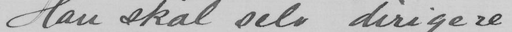Han skal selv dirigere.
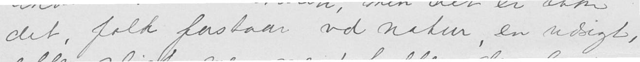det, folk forstaar ved natur, en udsigt,
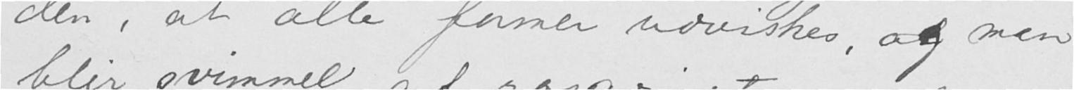den, at alle former udviskes, og man
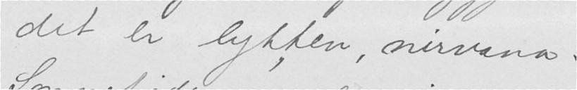det er lykken, nirvana.
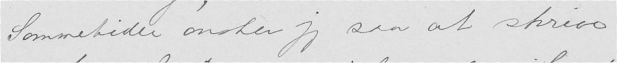Sommetider ønsker jeg saa at skrive
